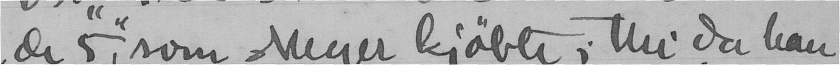"de 5", som Meyer "kjøbte"; thi da han
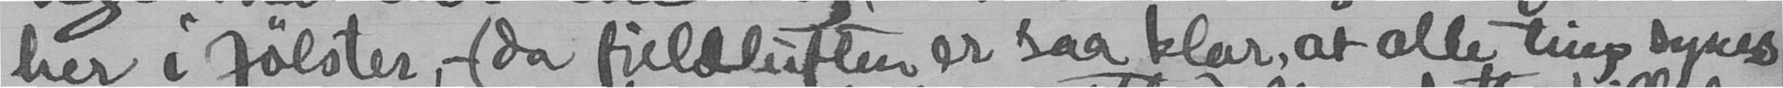her i Jølster, - (da fieldluften er saa klar, at alle ting synes
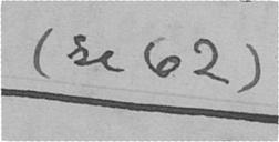(se 62)
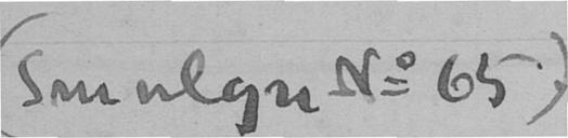(smnlgn No 65)
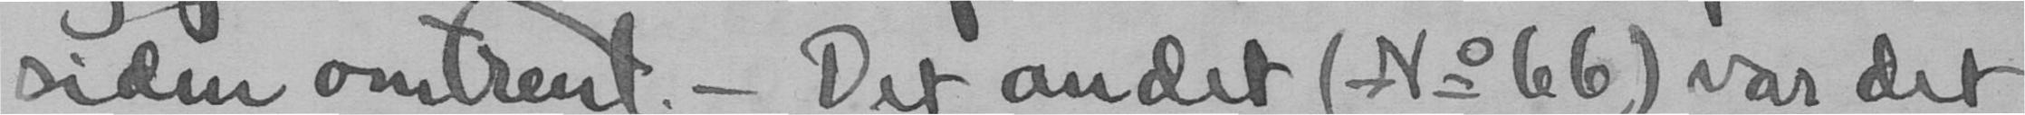siden omtrent. - Det andet (No 66) var det
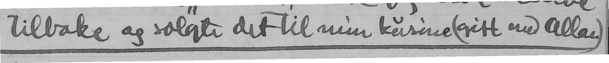tilbake og solgte det til min kusine (gitt med Allan)
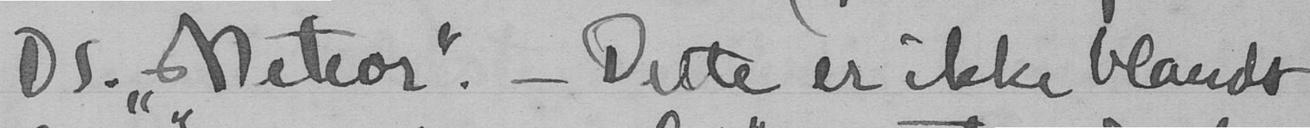Ds. "Meteor". - Dette er ikke blandt
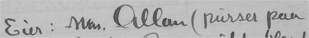Eier: Mtn. Allan (pusser paa
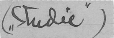("studie")
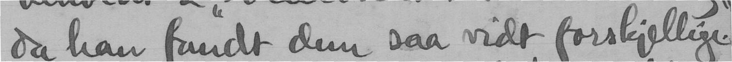da han fandt dem saa vidt forskjellige.
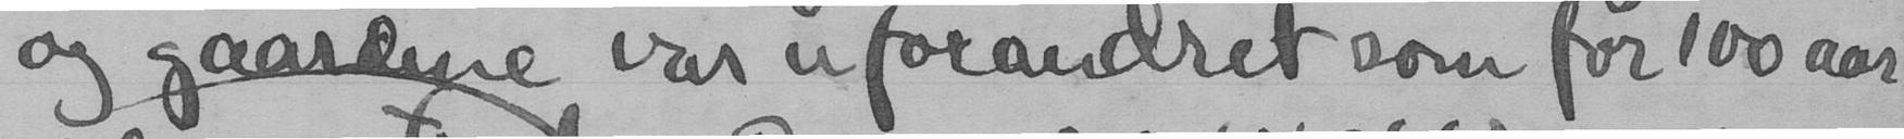og gaardene var uforandret som for 100 aar
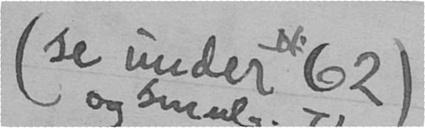(se under 62)
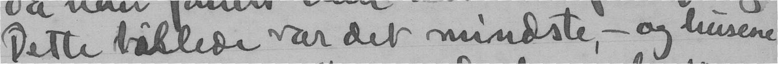Dette billede var det mindste, - og husene
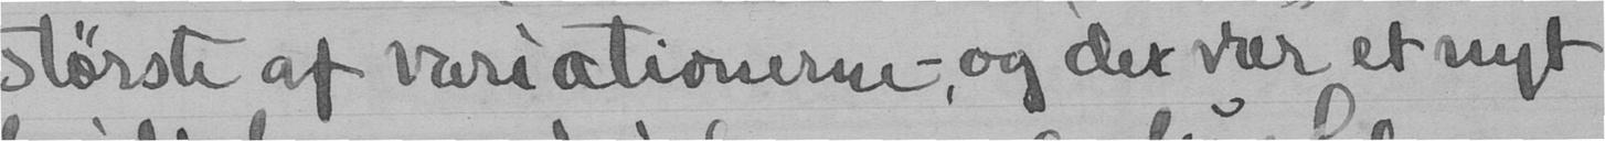største af variationerne -, og der var et nyt
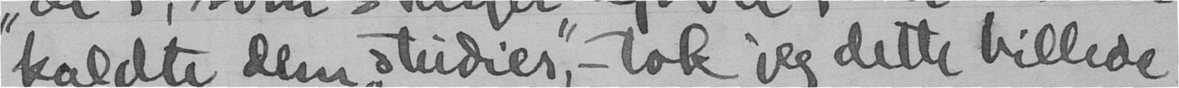kaldte dem "studier", - tok jeg dette billede
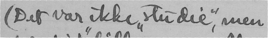(Det var ikke "studie", men
